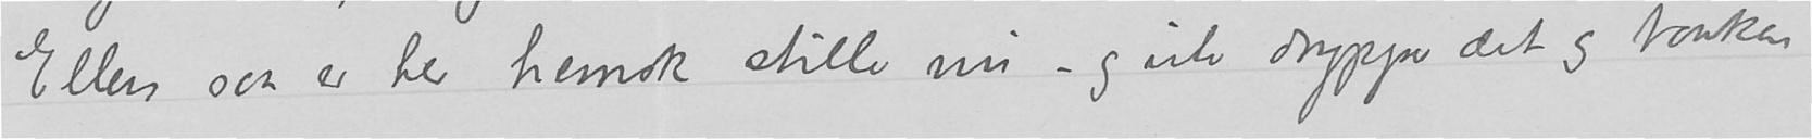Ellers saa er her hemsk stille nu – og ute drypper det og taaken
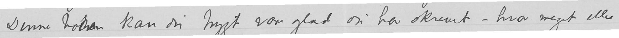Denne boken kan du trygt være glad du har skrevet – hvor meget eller
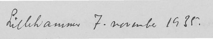Lillehammer 7. november 1935.
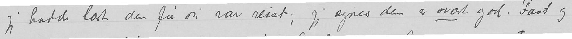jeg hadde læst den før du var reist; jeg synes den er svært god. Fast og
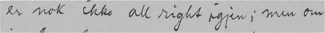er nok ikke all right igjen; men om
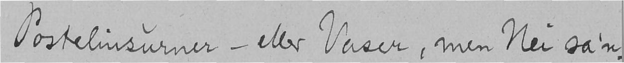Postelinsurner- eller Vaser, men Nei sa'n.
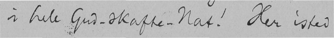i hele Gud-skafte-Nat! Her isted
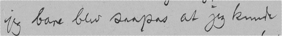jeg bare blev saapas at jeg kunde
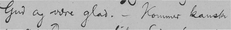Gud og være glad. - Kommer kanske
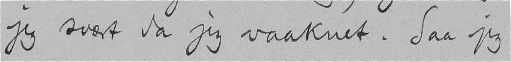jeg svært da jeg vaaknet. Saa jeg
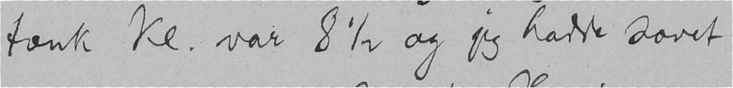tænk Kl. var 8 1/2 og jeg hadde sovet
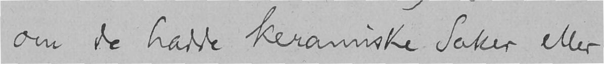om de hadde keramiske Saker eller
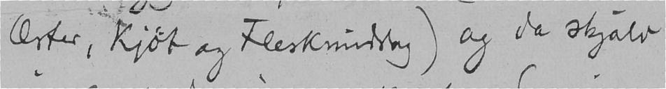Ærter, Kjøt og Fleskmiddag) og da skjalv
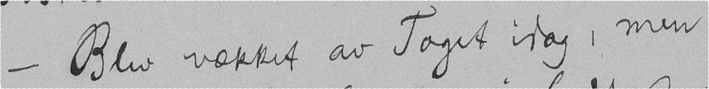- Blev vækket av Toget idag, men
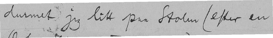durmet jeg litt paa Stolen (efter en
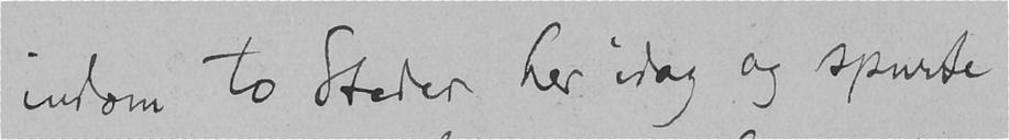indom to Steder her idag og spurte
